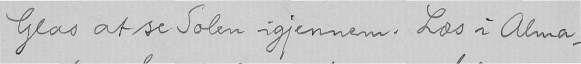Glas at se Solen igjennem. Læs i Alma-
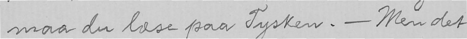maa du læse paa Tysken. - Men det
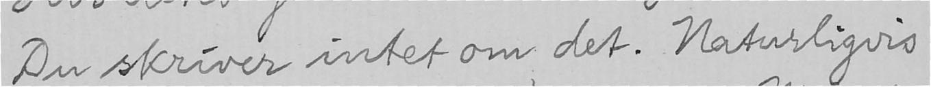Du skriver intet om det. Naturligvis
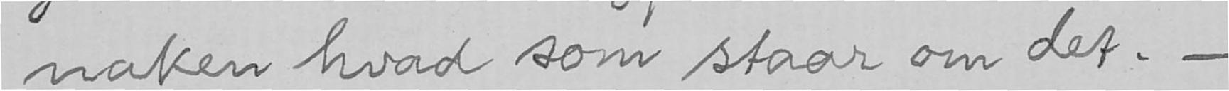naken hvad som staar om det. -
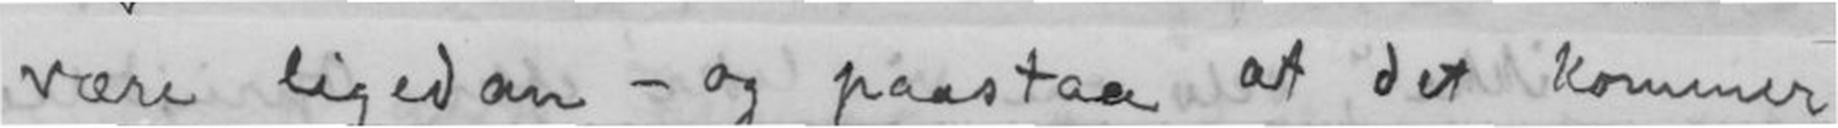være ligedan - og paastaa at det kommer
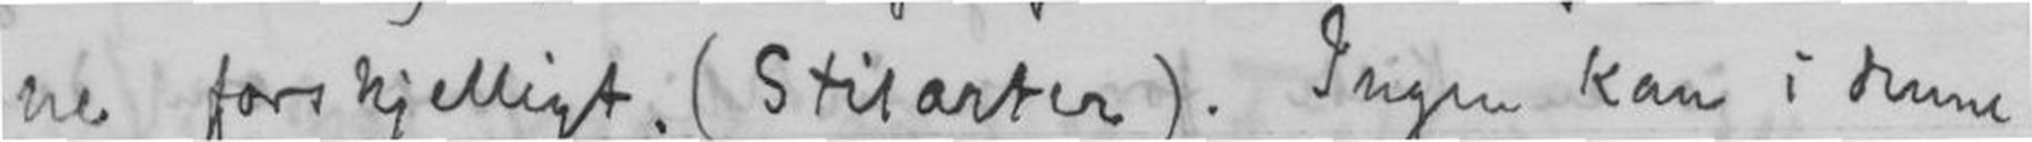ne forskjelligt. (Stilarter). Ingen kan i denne
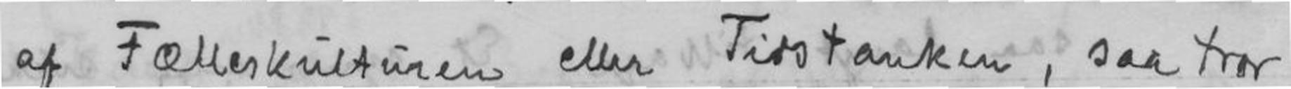af Fælleskulturen eller Tidstanken, saa tror
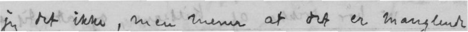jeg det ikke, men mener at det er manglende
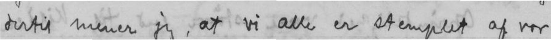dertil mener jeg, at vi alle er stemplet af vor
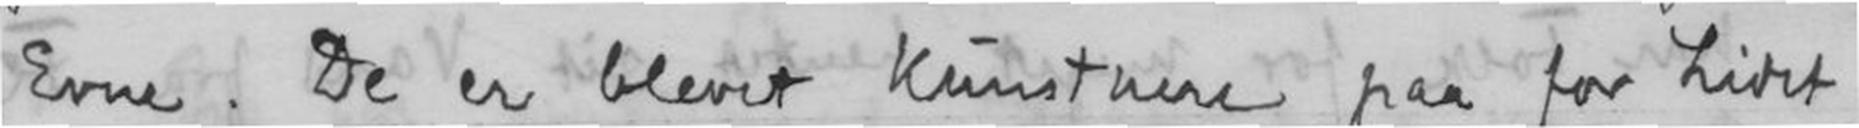Evne. De er blevet Kunstnere paa for Lidet
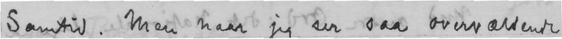Samtid. Men naar jeg ser saa overvældende
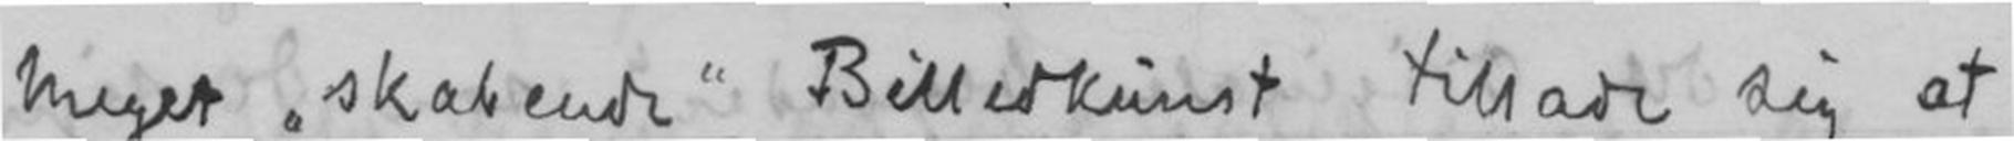meget skabende Billedkunst tillade sig at
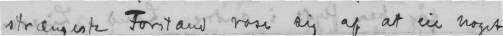strængeste Forstand rose sig af at eie noget
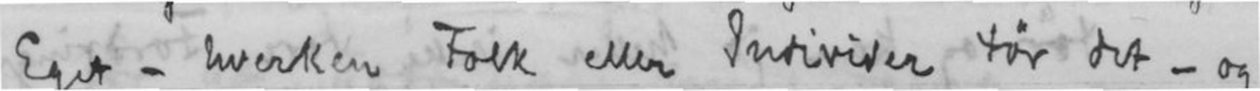Eget - hverken Folk eller Individer tør det - og
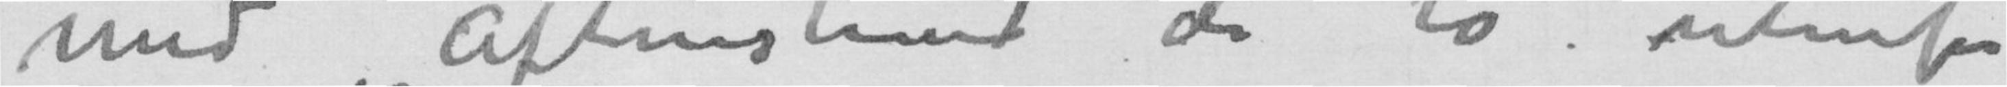med Aftenstund de to utenfor
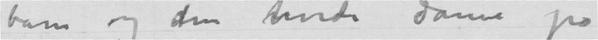barn og den hvide dame på
